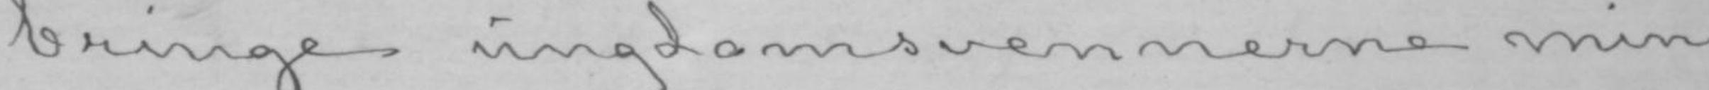bringe ungdomsvennerne min
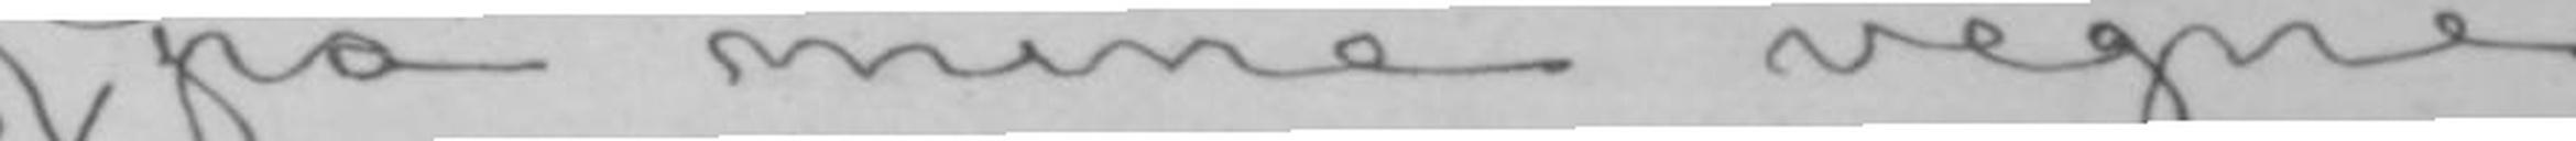på mine vegne
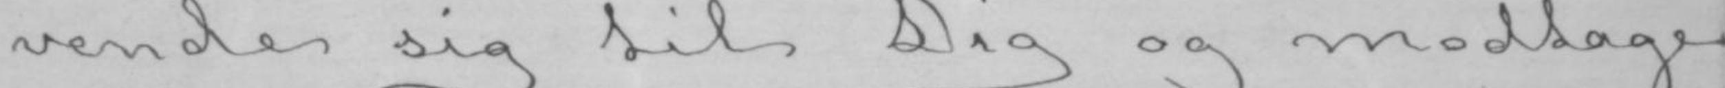vende sig til Dig og modtage
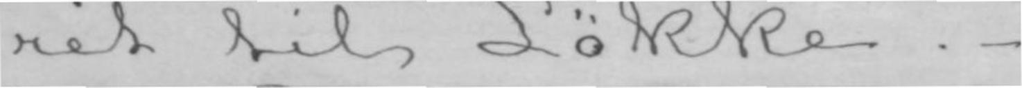ret til Løkke.-
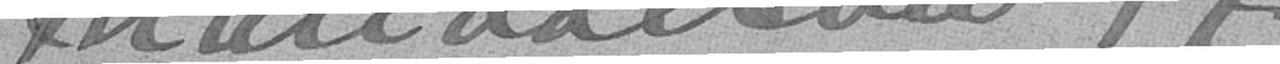Maridalsvei 17
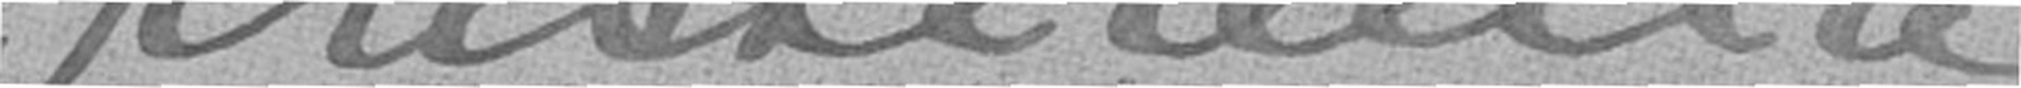Kristiania
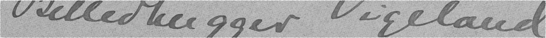Billedhugger Vigeland
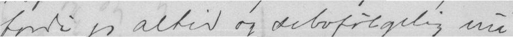fordi jeg altid og selvfølgelig nu
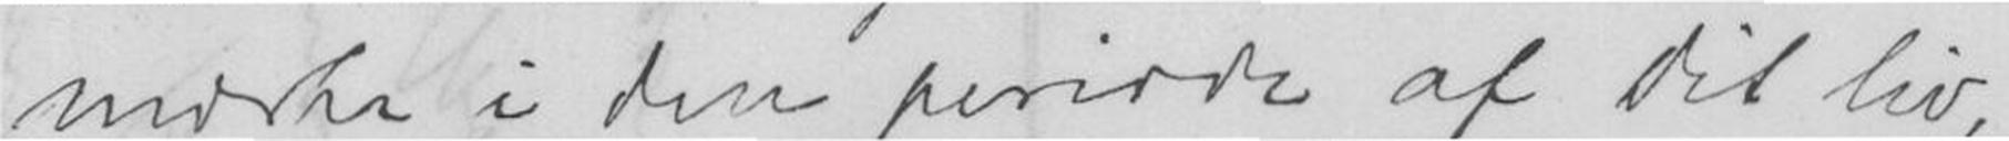mærke i den periode af dit liv,
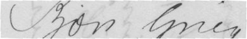Kjære Grieg!
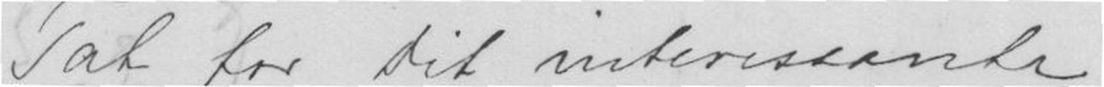Tak for dit interessante
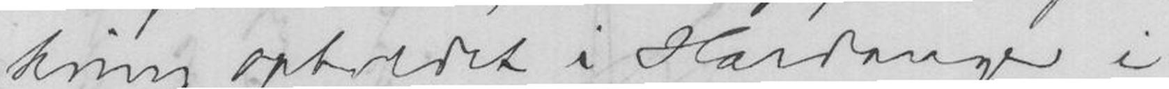kring opholdet i Hardanger i
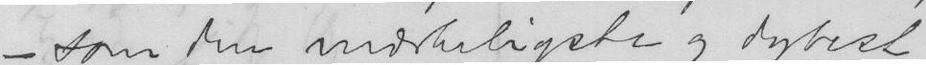som den mærkeligste og dybest
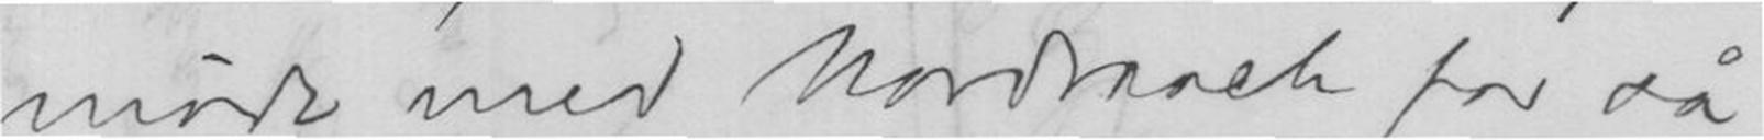møde med Nordaack for så
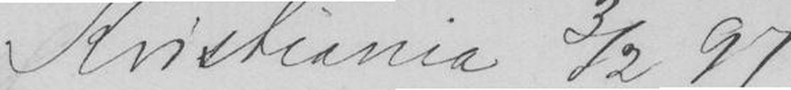Kristiania 3/2 97
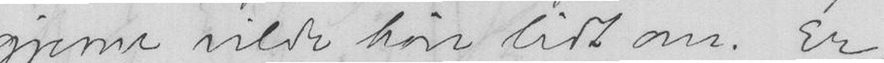gjerne vilde høre lidt om. Er,
